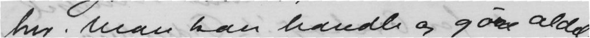her. Man kan handle og gøre aldeles
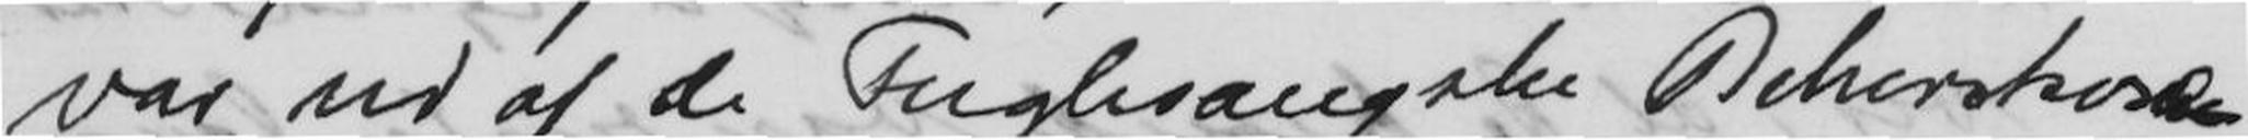var ud af de Fuglesangske Behorskosen
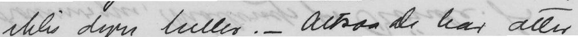ikke dyre heller. - Altsaa De har atter
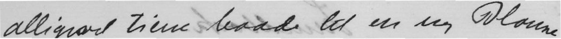alligevel tiene baade til en ny Blouse
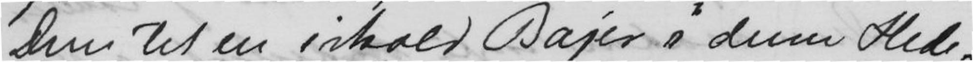Dem til en iskald Bajer i denne Hede!
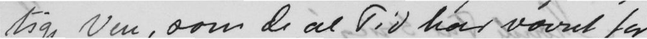tige Ven, som De al Tid har vaeret for
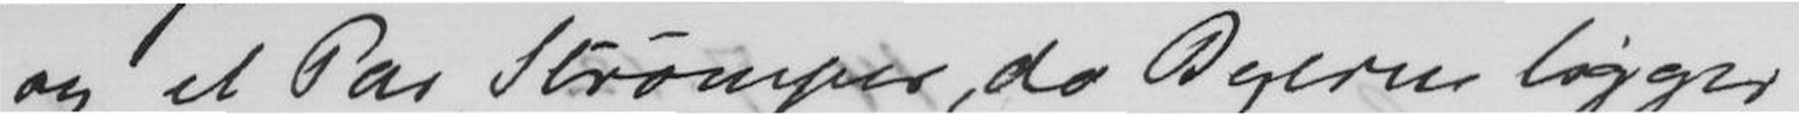og et Par Strømper, da Byerne ligger
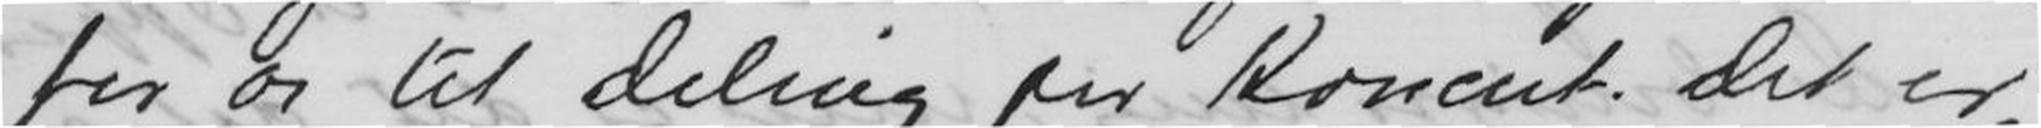for os til deling per Koncert. Det er
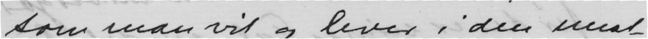som man vil og lever i den mest
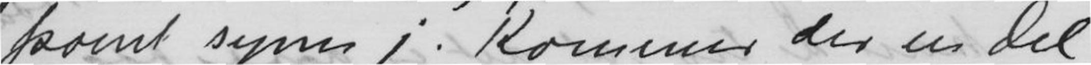paent synes j. Kommer der en Del
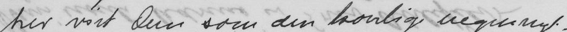her vist Dem som den koselig uegennyt-
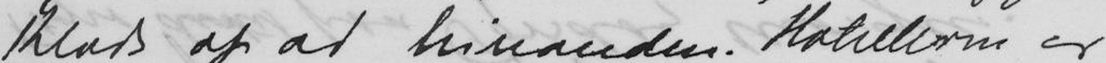Klods op ad hinanden. Hotelrom er
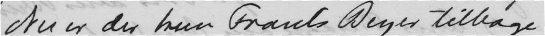Nu er der kun Frants Beyer tilbage,
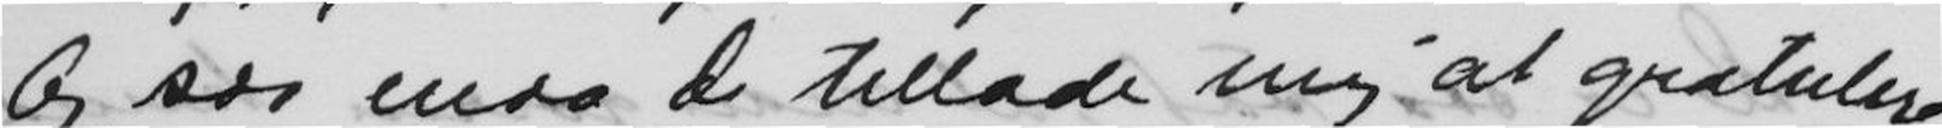Og saa enda De tillade mig at gratulere
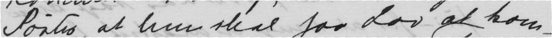Søster, at hun skal faa Lov at kom-
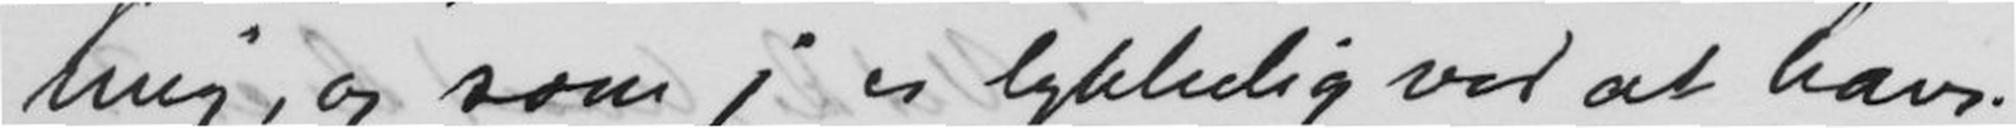mig, og som j er lykkelig ved at have.
